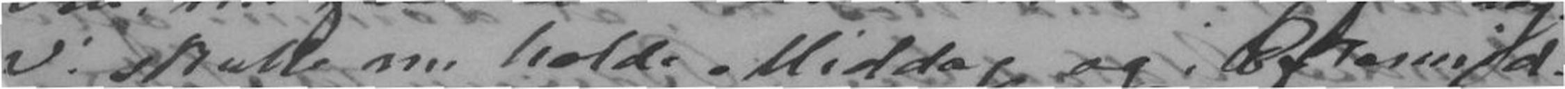Vi skulde nu holde Middag og i Eftermid-
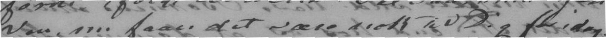Ven, nu faaer det være nok til Dig for idag.
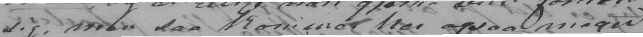sig, men saa kommer her ogsaa meget
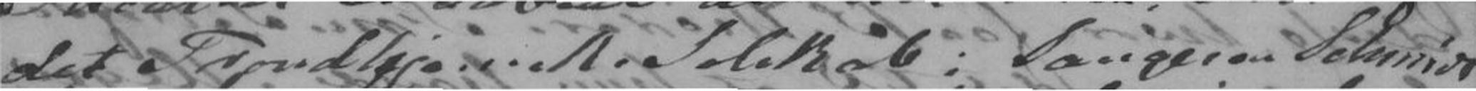det Trondhjemske Selskab; Sangeren Schmidt
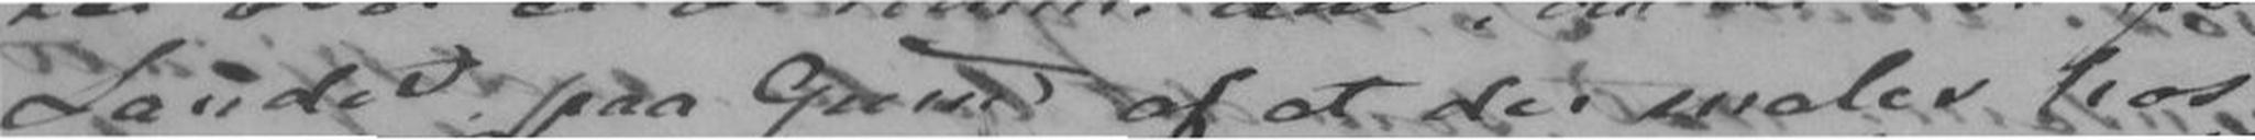Landet paa Grund af at det males hos
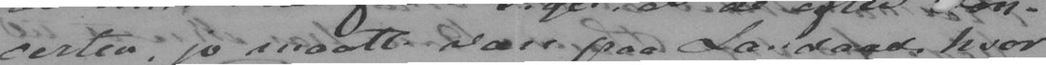certen, jo maatte være paa Landaas hvor
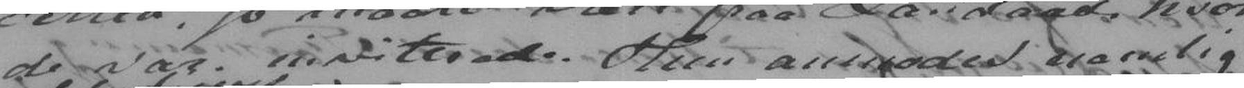de var inviterede. Hun anmoder nemlig
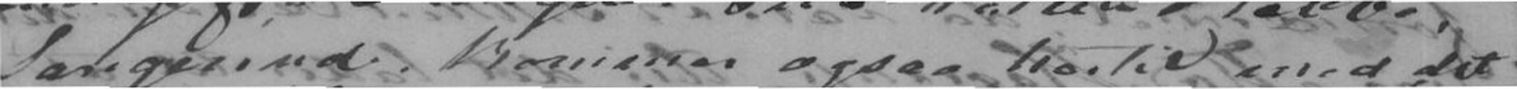Sangerinde kommer ogsaa hertil, med det
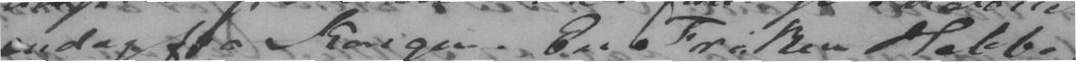endog fra Kongen. En Frøken Habbe,
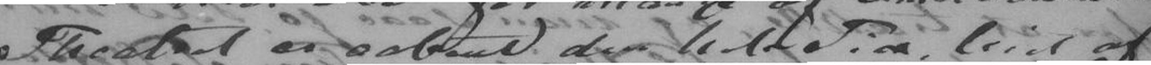Theatret er aabent den hele Tid, leiet af
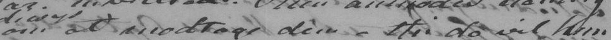Scherdiwys om at modtage dem, thi da vil hun
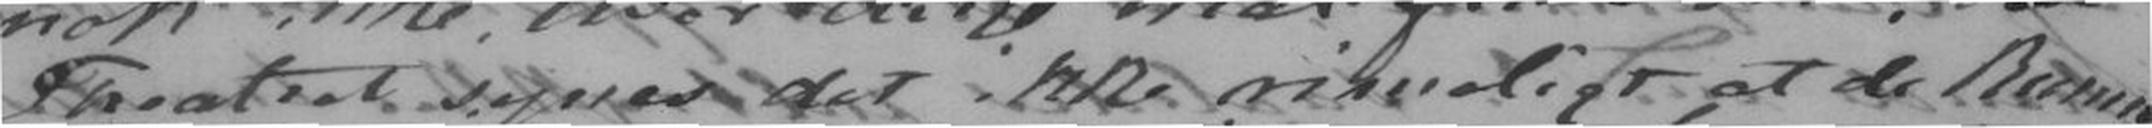Theatret synes det ikke rimeligt at de kunne
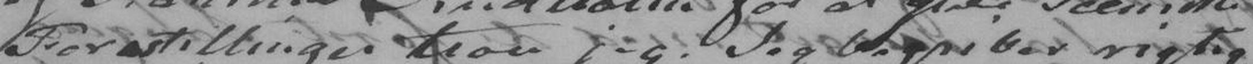Forestillinger troer jeg. Jeg begriber rigtig-
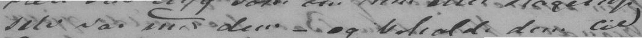selv var med dem - og beholde dem til
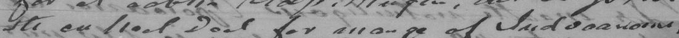ste en heel Deel for mange af Indvaanerne.
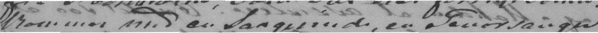kommer med en Sangerinde, en Tenorsanger
